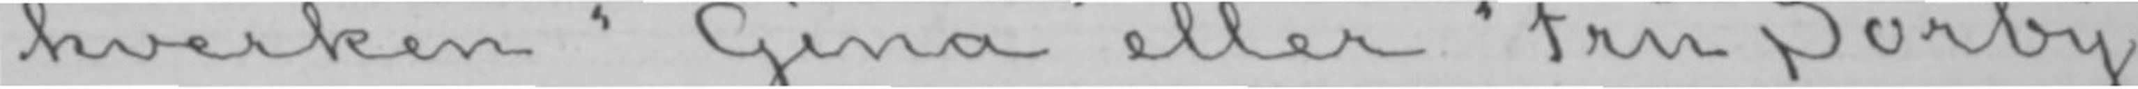hverken «Gina» eller «Fru Sørby»
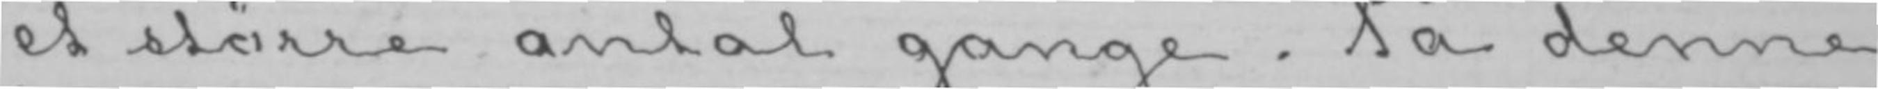et større antal gange. På denne
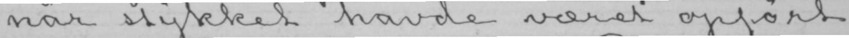når stykket havde været opført
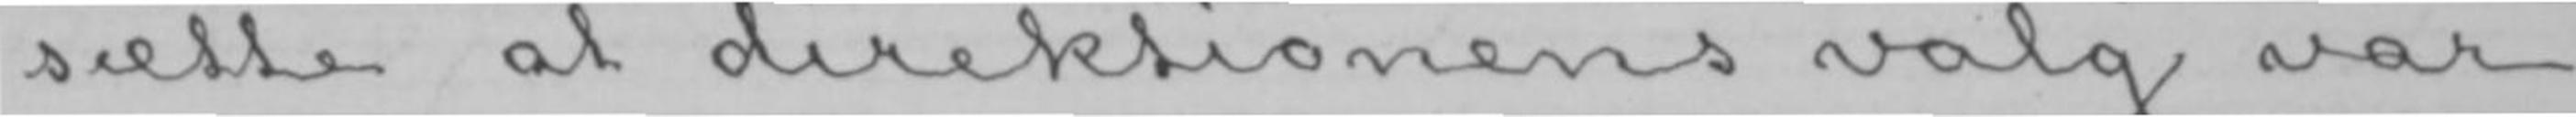sætte at direktionens valg var
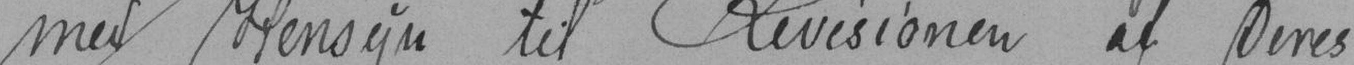med Hensyn til Revisionen af Deres
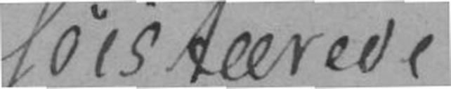Høistærede
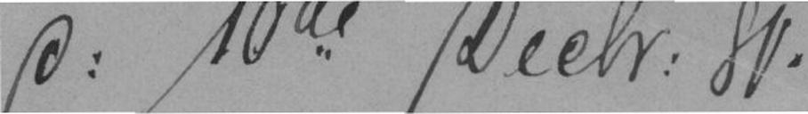10de Decbr. 81
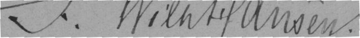S. Wilhelm Hansen
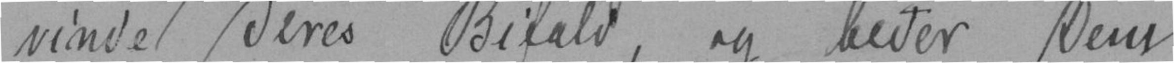vinde Deres Bifald, og beder Dem
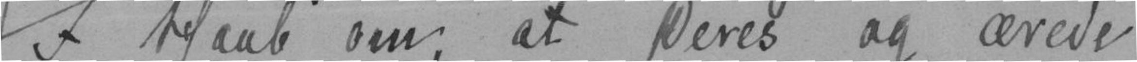I Haab om, at Deres og ærede
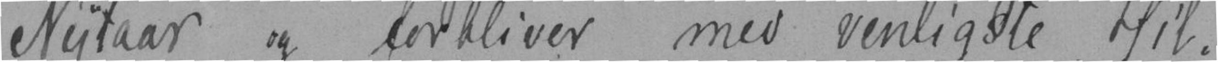Nytaar og forbliver med venligste Hil-
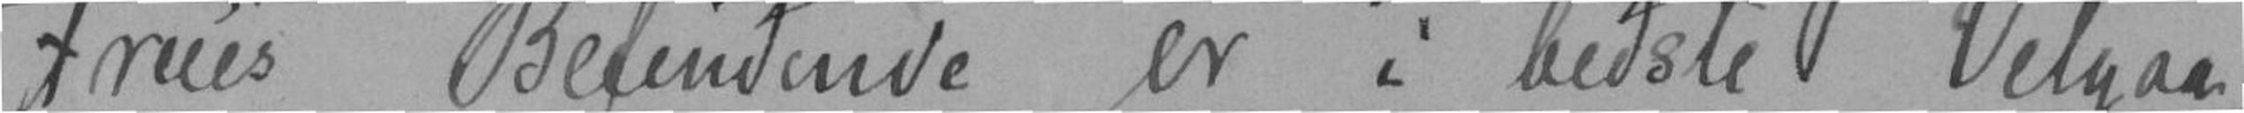Fruens Befindende er i bedste Velgaa-
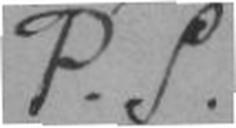P.S.
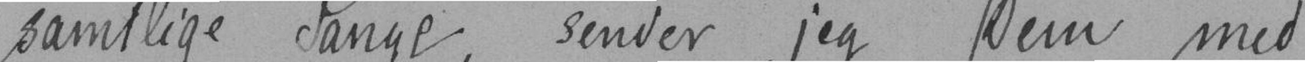samtlige Sanger, sender jeg Dem med
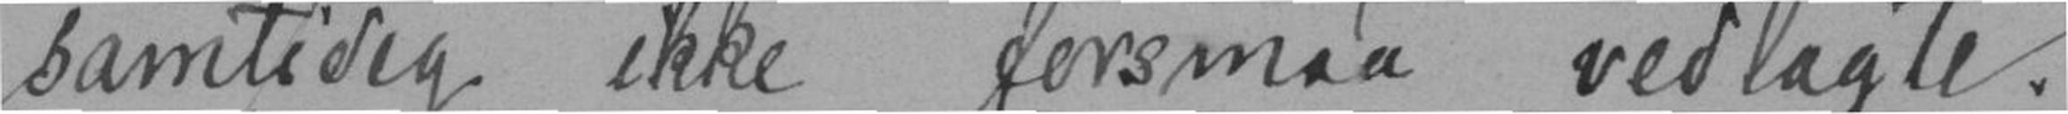samtidig ikke forsmaa vedlagte.
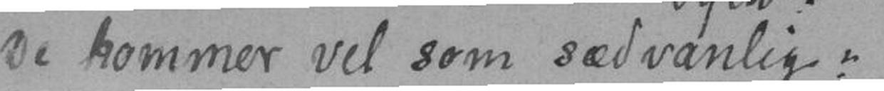De kommer vel som sædvanlig
